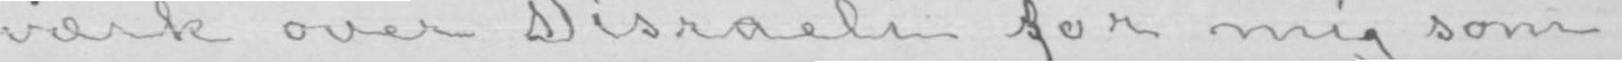værk over Disraeli sfor mig som
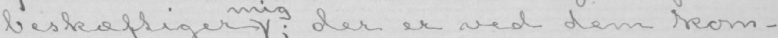beskæftiger mig; der er ved dem kom-
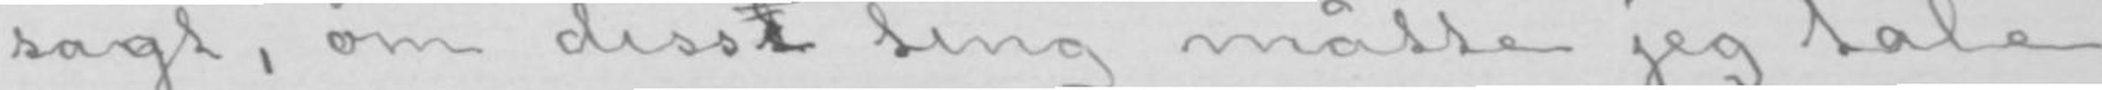sagt, om disste ting måtte jeg tale
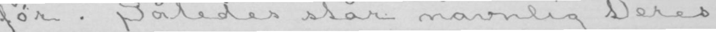før. Således står navnlig Deres
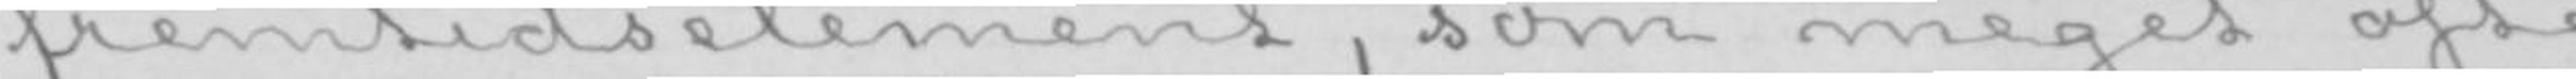fremtidselement, som meget ofte
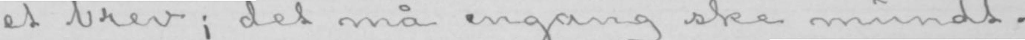et brev; det må engang ske mundt-
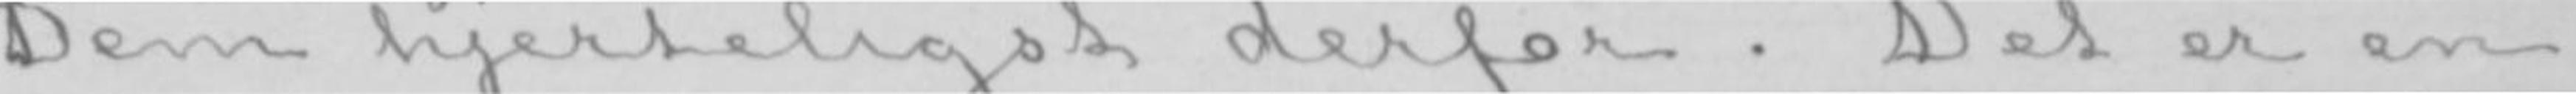Dem hjerteligst derfor. Det er en
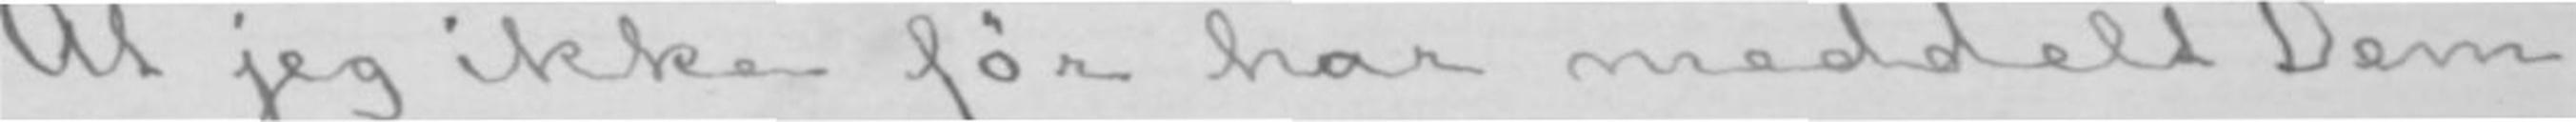At jeg ikke før har meddelt Dem
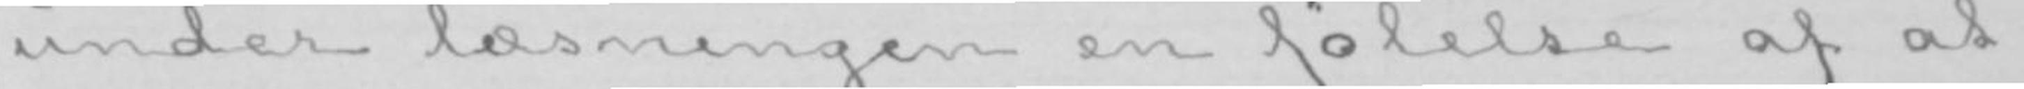under læsningen en følelse af at
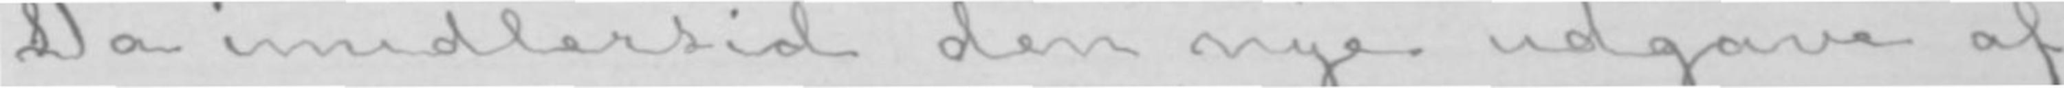Da imidlertid den nye udgave af
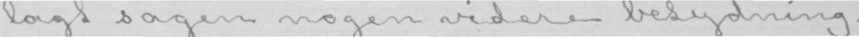lagt sagen nogen videre betydning.
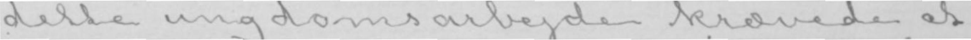dette ungdomsarbejde krævede et
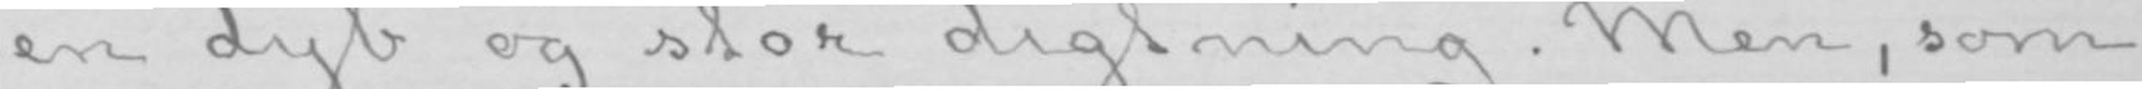en dyb og stor digtning. Men, som
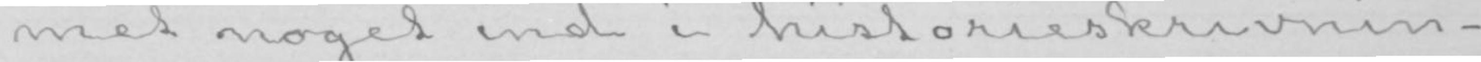met noget ind i historieskrivnin-
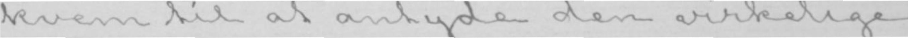kvem til at antyde den virkelige
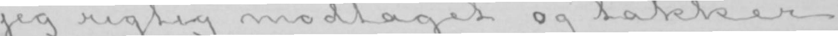jeg rigtig modtaget og takker
